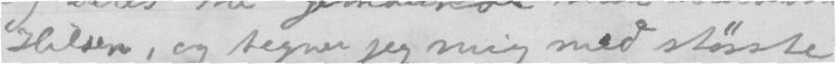Hilsen, og tegner jeg mig med største
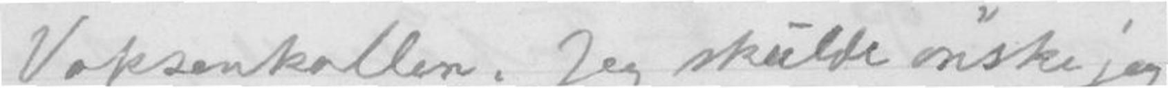Voksenkollen. Jeg skulde ønske jeg
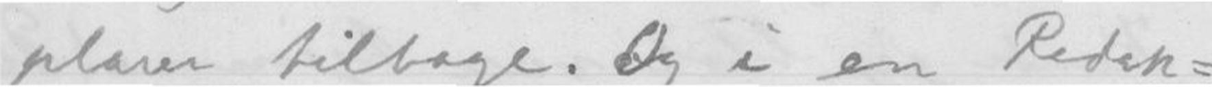plarer tilbage. Og i en Redak-
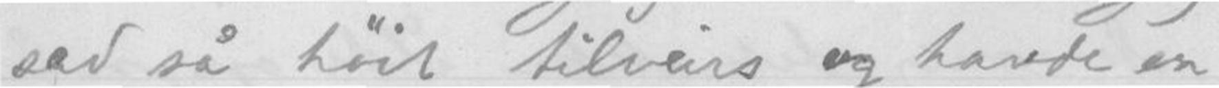sad så høit tilveirs og havde en
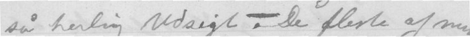så herlig Udsigt. De fleste af mine
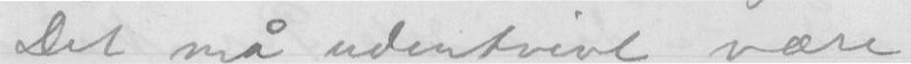Det må udentvivl være
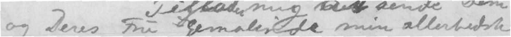og Deres Fru Gemalinde min allerbedste
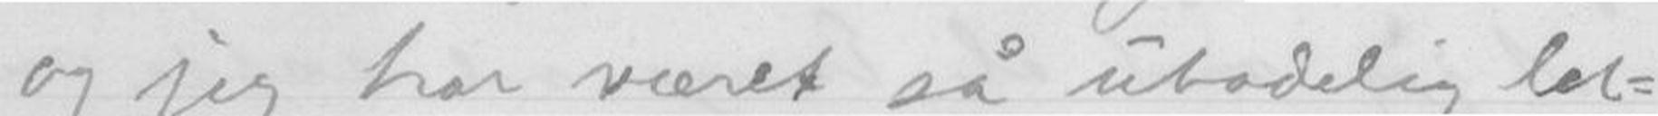og jeg har været så ubodelig let-
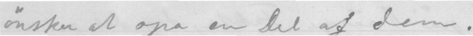ønsker at opa en Del af dem,
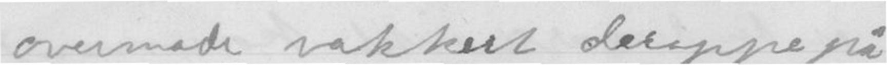overmåde vakkert deroppe på
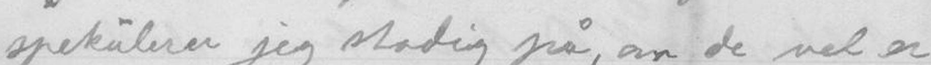spekulerer jeg stadig på, om de vel er
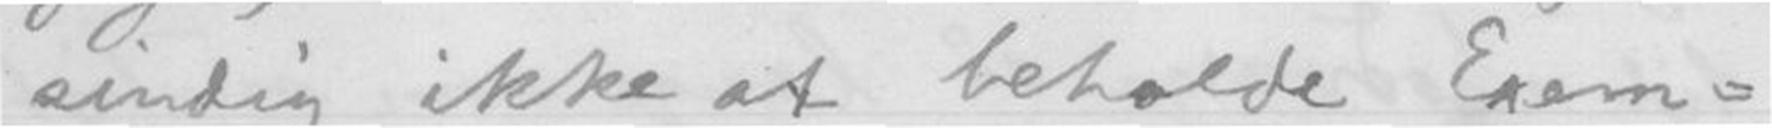sindig ikke at beholde Exem-
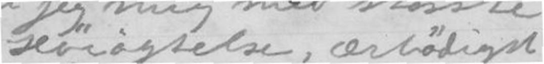Høiagtelse, ærbødigst
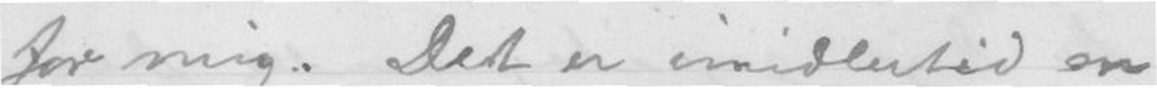for mig. Det er imidlertid en
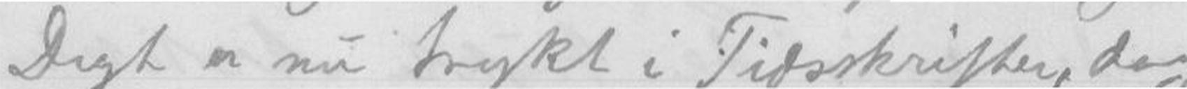Digt er nu trykt i Tidskrifter, dog
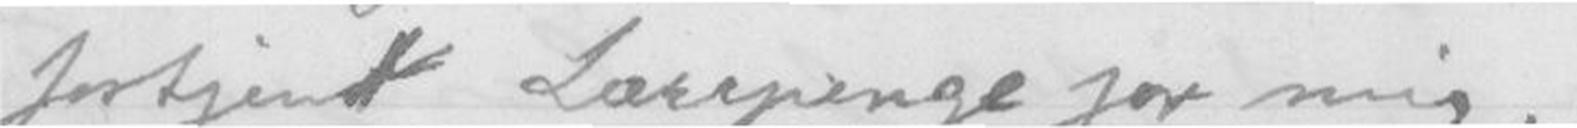fortjent Lærepenge for mig. -
